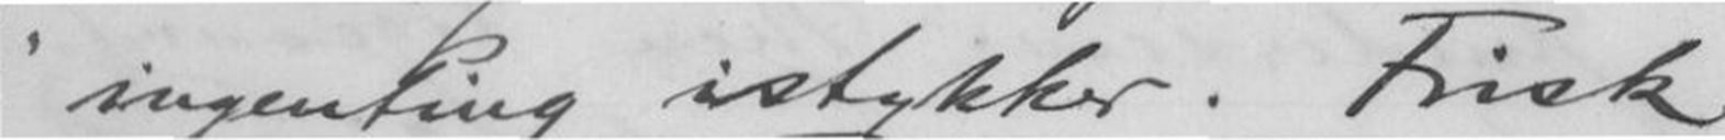ingenting istykker. Frisk
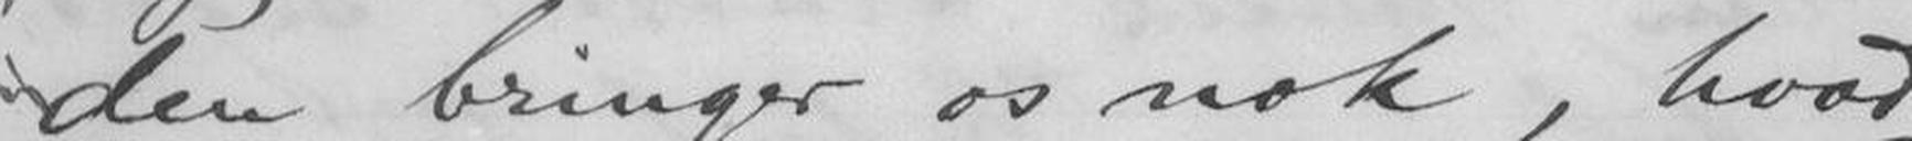den bringer os nok,
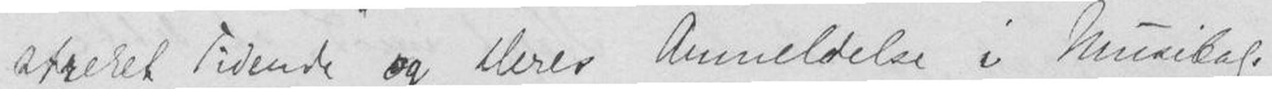streret Tidende" og Deres Anmeldelse i Musikal.
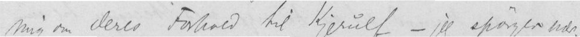mig om deres Forhold til Kjerulf - jeg spørger nær-
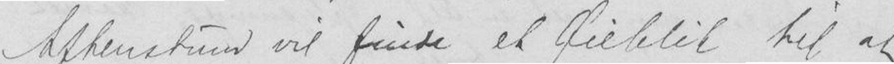Aftenstund vil finde et Øieblik til at
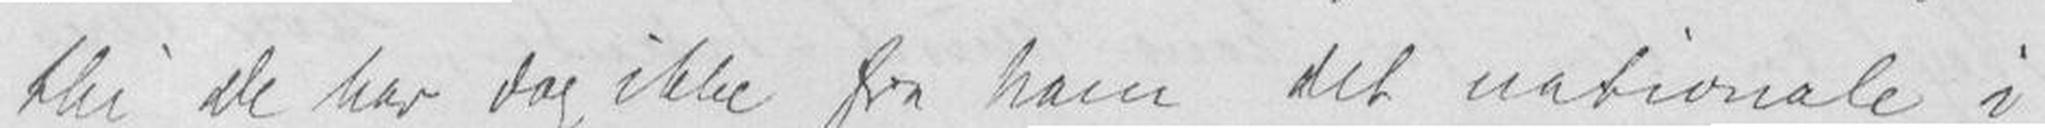thi de har dog ikke fra ham det nationale i
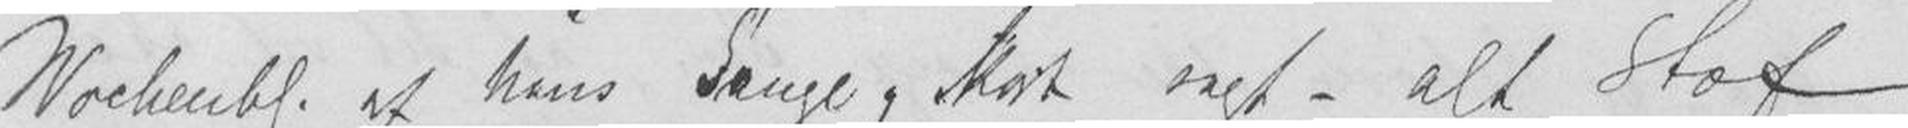Wochenbl. af hans Sange, kort sagt - alt Stof
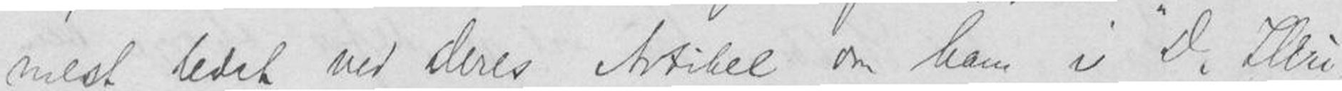mest ledet ved deres Artikel om ham i D.Illu-
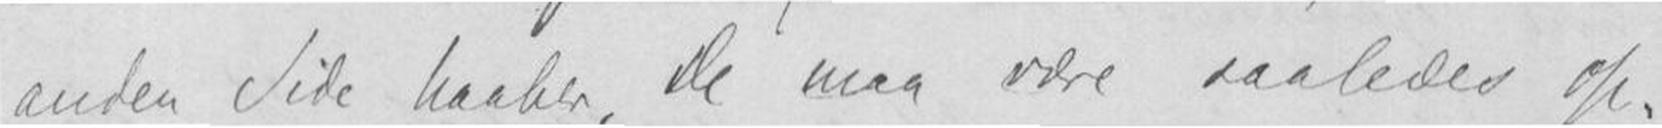anden Side haaber, De maa være saaledes op-
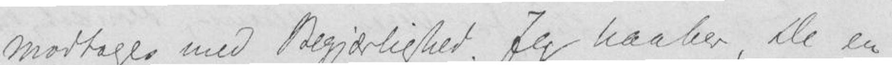mottager med Begjærlighed. Jeg haaber, De en
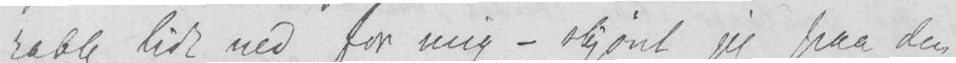rable ned for mig - skjønt jeg paa den
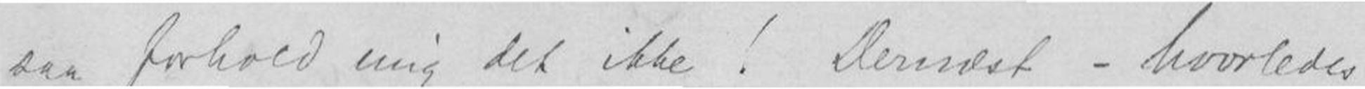saa forhold mig det ikke! Dernæst - hvorledes
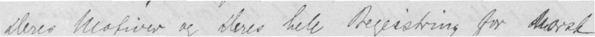Deres Motivert og Deres hele Begeistring for Norsk
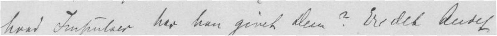hvad Inpulser har han givet Dem? Er det andet
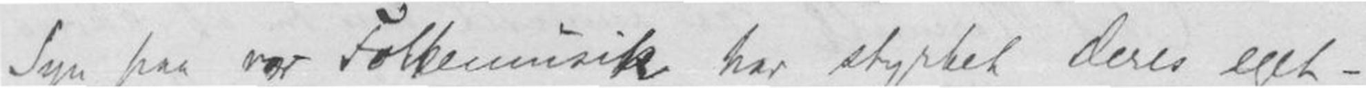Syn paa vor Folkemusik har styrket Deres eget -
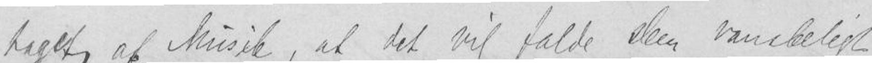taget, af Musik, at det vil falde Dem vanskelig
